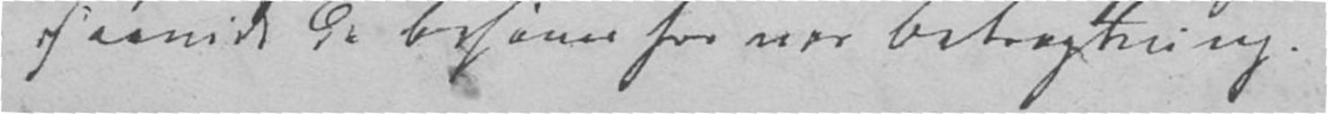saavidt de behøves for vor Betragtning.
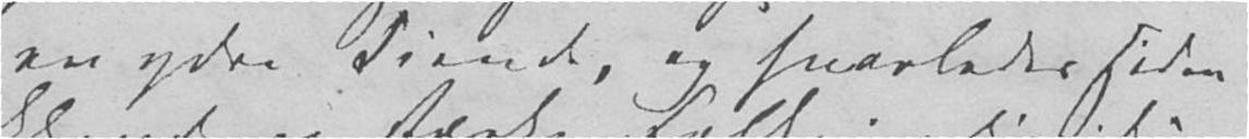en ydre Fiende, og hvorledes siden
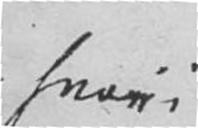hvori
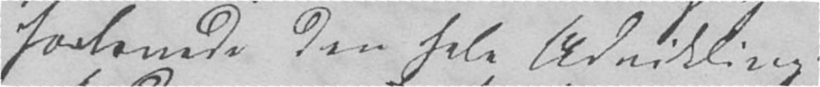forlenede den hele Udvikling
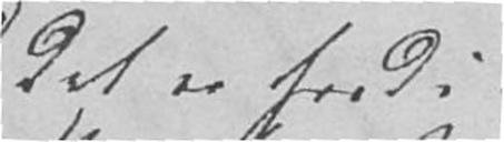Det er fordi
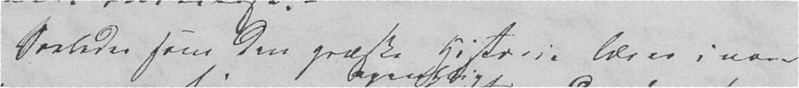Saaledes som den græske Historie læres i vore
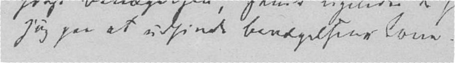søg paa at udfinde Bevægelsens Love.
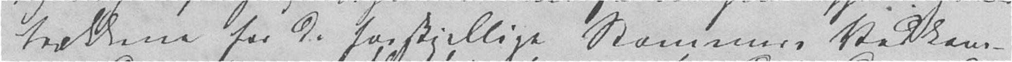trækkene for de forskjellige Stammers Vedkom-
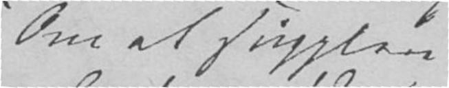Om at supplere
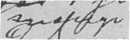mange
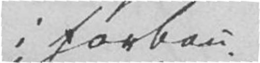i forbau-
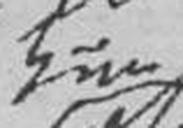kun
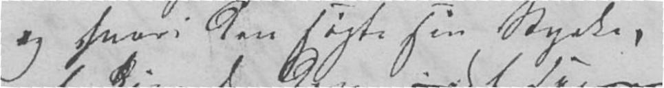og hvori den søgte sin Styrke,
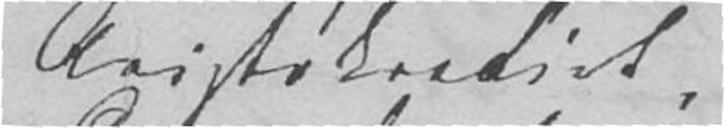Aristokratiet,
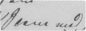Scene med
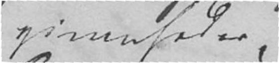givenheder,
